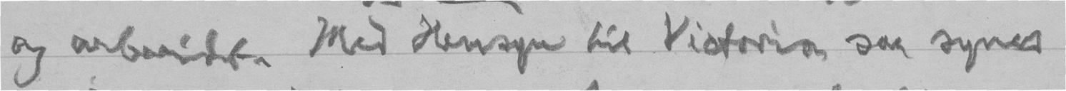og arbeidet. Med Hensyn til Victoria saa synes
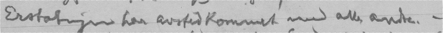Erstatningen har avstedkommet med alle andre. -
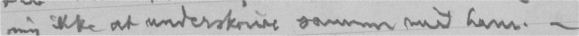mig ikke at underskrive sammen med ham. -
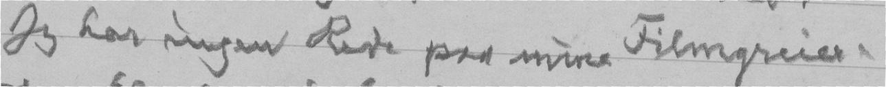Jeg har ingen Rede paa mine Filmgreier.
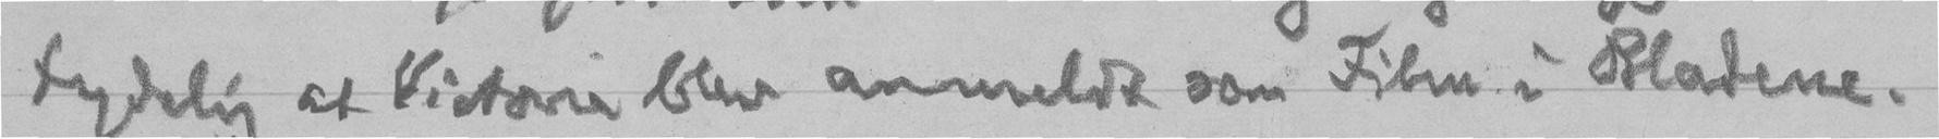tydelig at Victoria blev anmeldt som Film i Bladene.
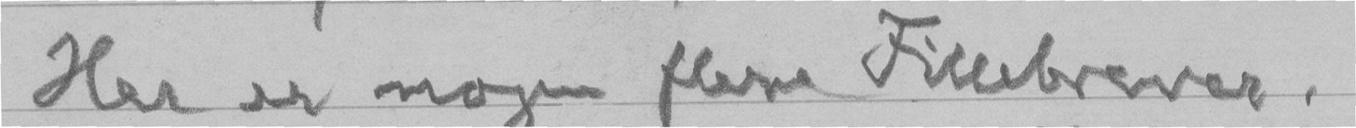Her er nogen flere Fillebrever.
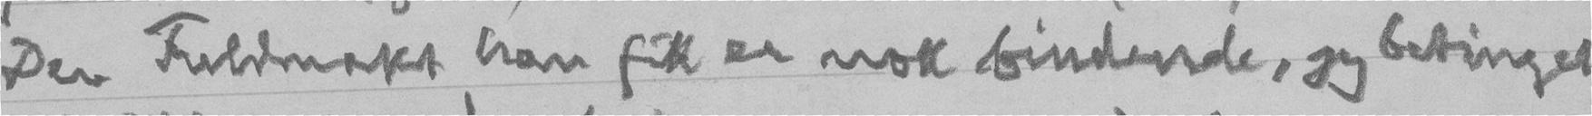Den Fuldmakt han fik er nok bindende, jeg betinget
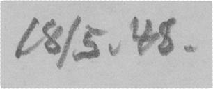18/5. 48.
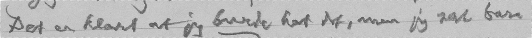Det er klart at jeg burde hat det, men jeg sat bare
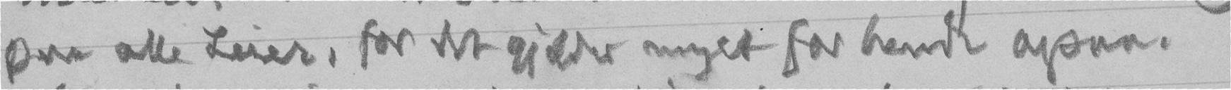paa alle Leier, for det gjelder meget for hende ogsaa.
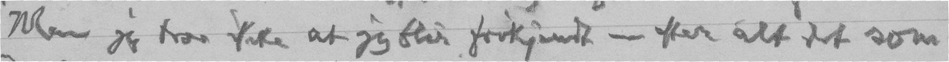Men jeg tror ikke at jeg blir frikjendt - efter alt det som
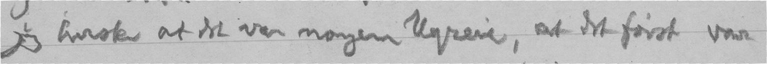jeg huske at det var nogen Ugreie, at det først var
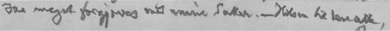saa meget forgjæves med mine Saker. - Hilsen til dere alle,
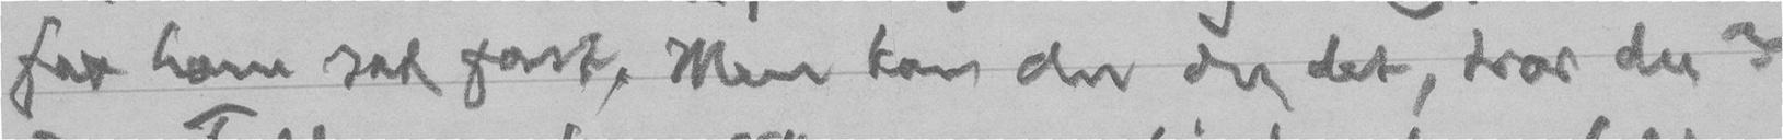faa ham sat fast. Men kan du du det, tror du?
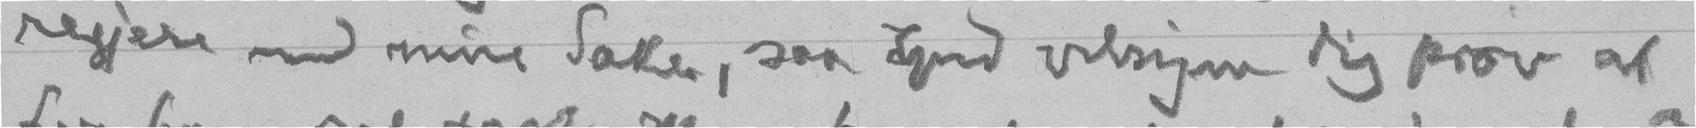regjere med mine Saker, saa Gud velsigne dig prøv at
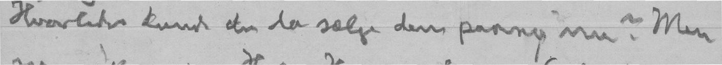Hvorledes kunde du da sælge den paany nu? Men
